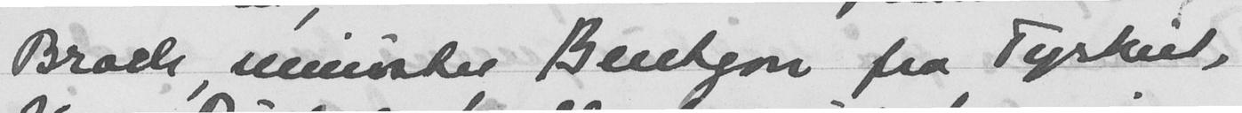Broch, minister Bentgon fra Tyskiel,
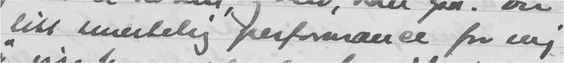litt merkelig performance for mig
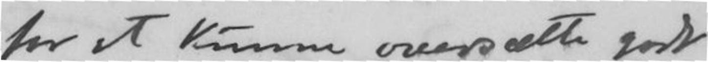for at kunne oversætte godt
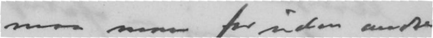maa man faa uden andre
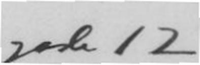gade 12
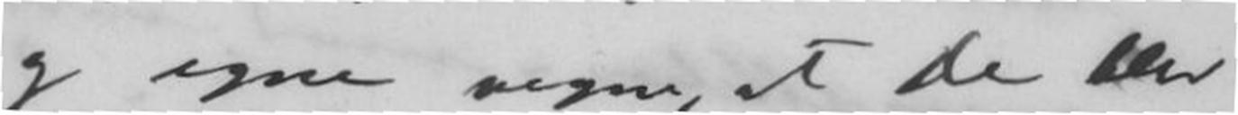og egne vegne, at De
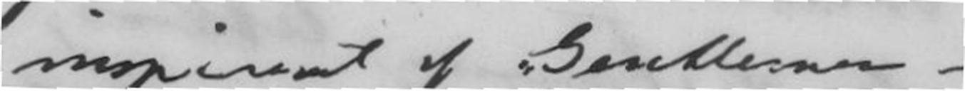inspireret af "Gentleman-
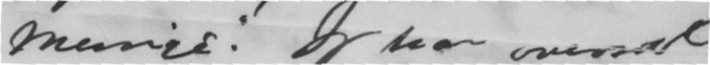musick: Jeg har oversat
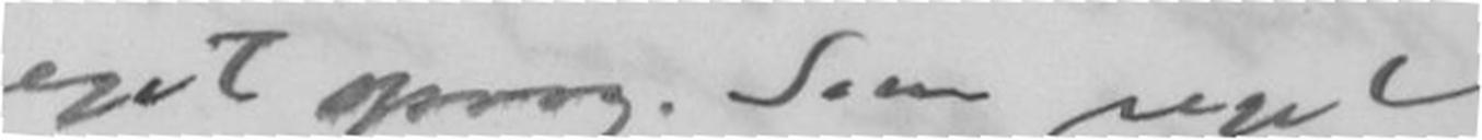eget sprog. Som regel
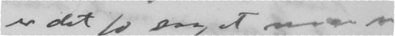er det jo saa, at noen i
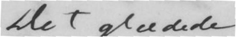Det glædede
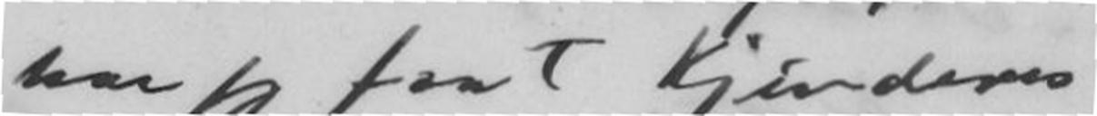har jeg faaet Kjenderes
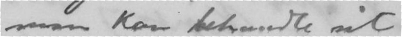man Kan behandle sit
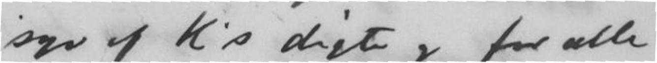syv af K's digt, for alle
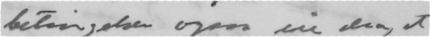betingelser ogsaa vide at
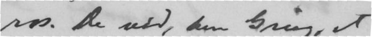ros. De ved, kun Grieg, at
